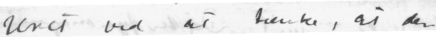Uret ved at tænke, at der
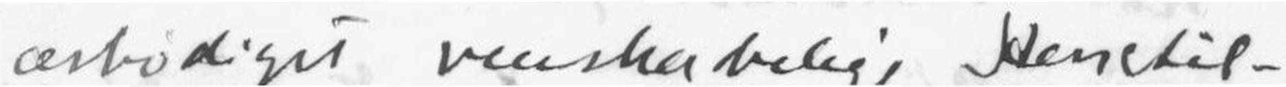Ærbødigst venskabeligt Henstil-
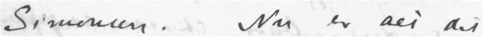Simonsen. Nu er alt det
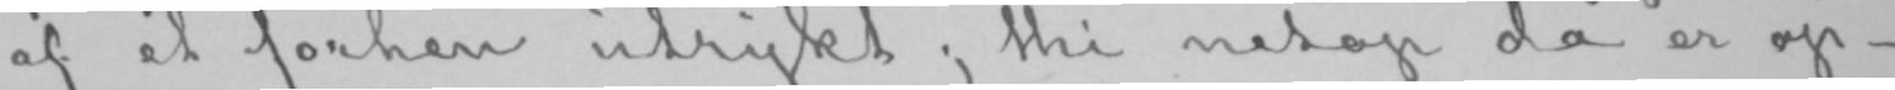af et forhen utrykt; thi netop da er op-
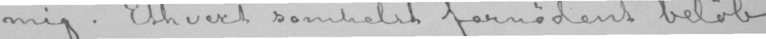mig. Ethvert somhelst fornødent beløb
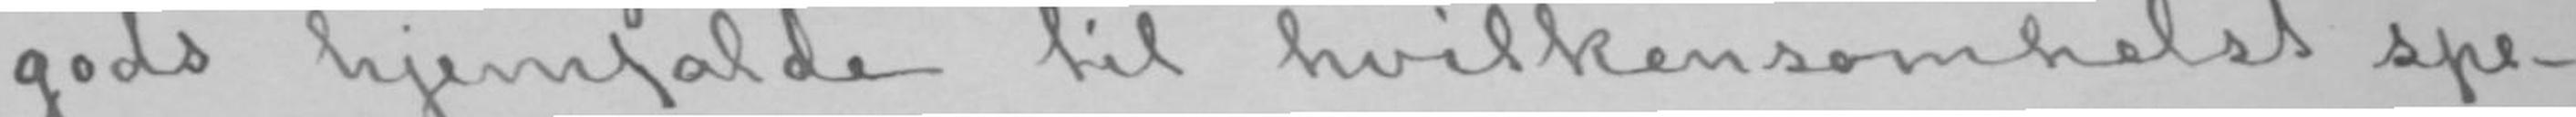gods hjemfalde til hvilkensomhelst spe-
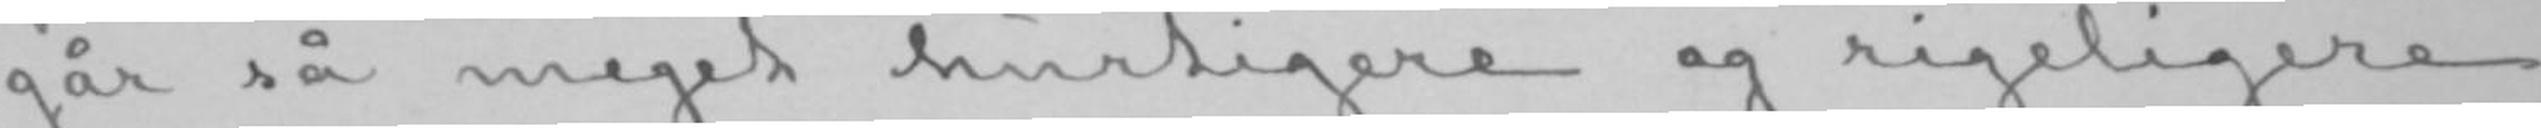går så meget hurtigere og rigeligere
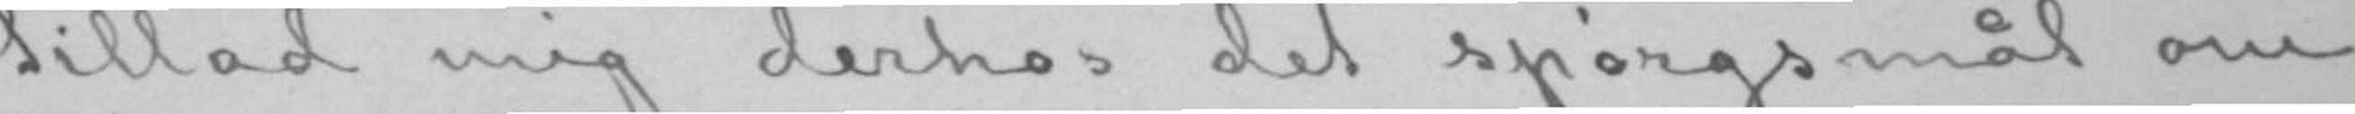Tillad mig derhos det spørgsmål om
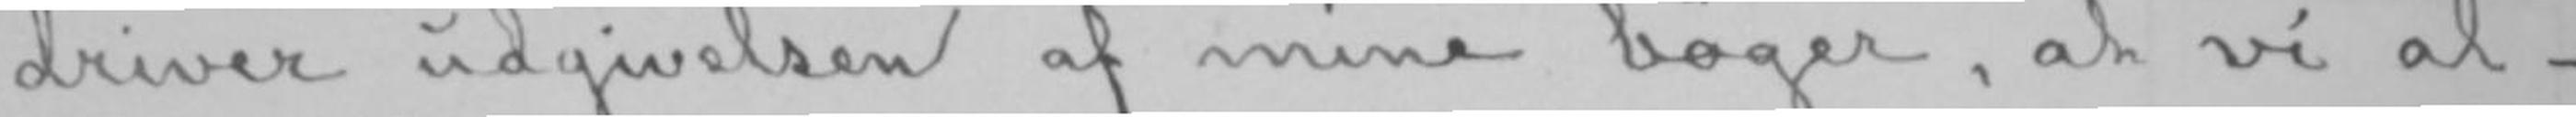driver udgivelsen af mine bøger, at vi al-
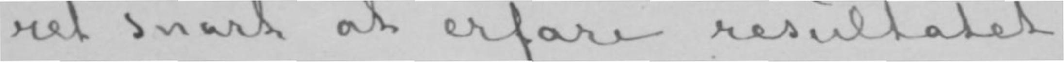ret snart at erfare resultatet.
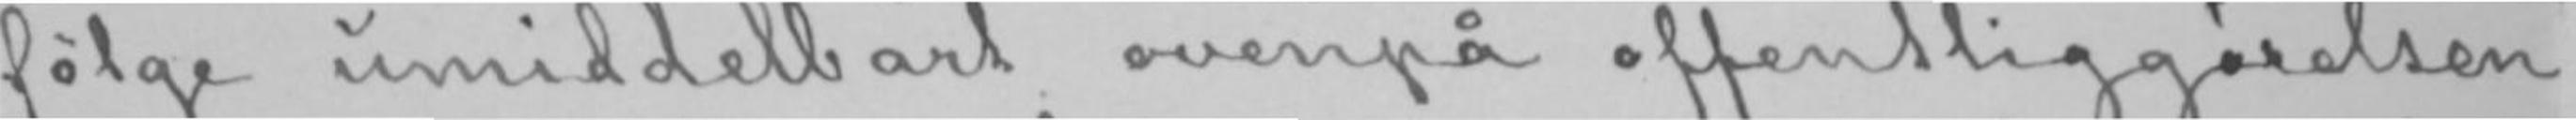følge umiddelbart ovenpå offentliggørelsen
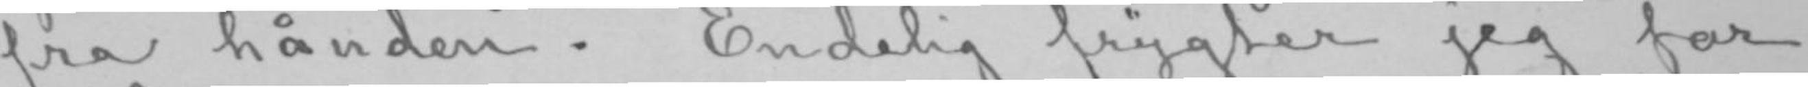fra hånden. Endelig frygter jeg for
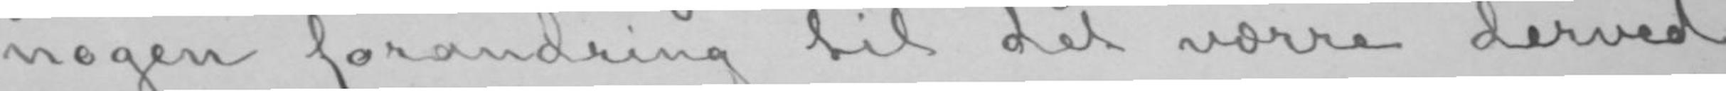nogen forandring til det værre derved
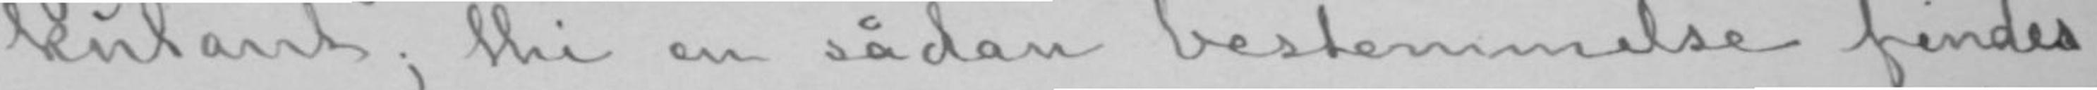kulant; thi en sådan bestemmelse findes
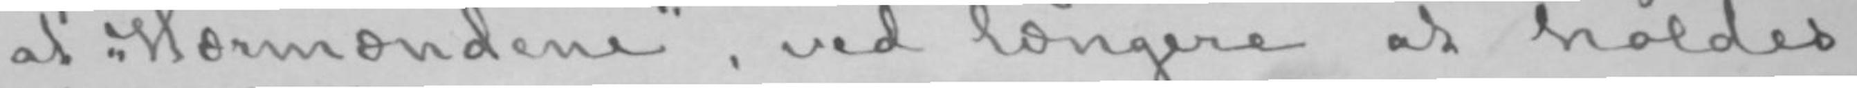at «Hærmændene», ved længere at holdes
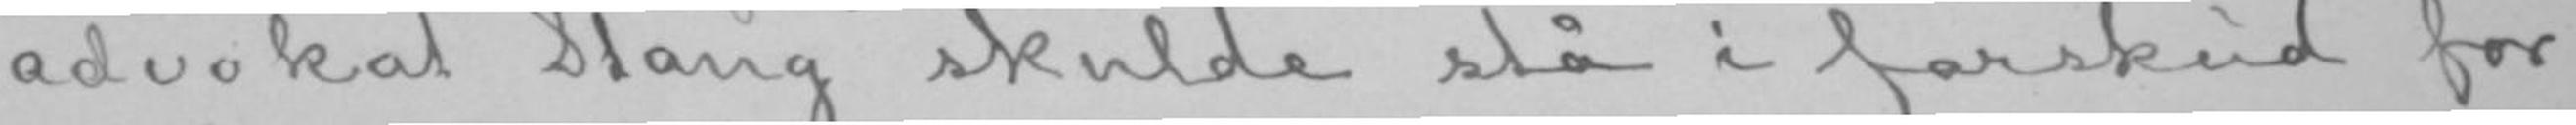advokat Stang skulde stå i forskud for
